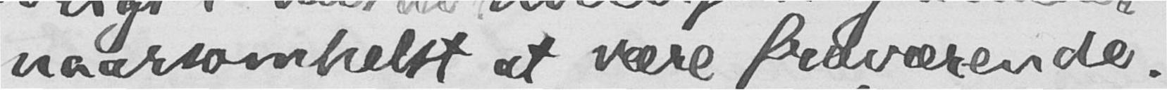naarsomhelst at være fraværende.
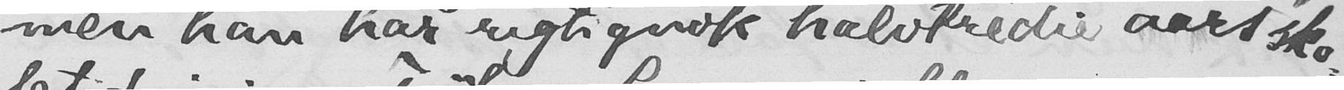men han har rigtignok halvtredie aars sko-
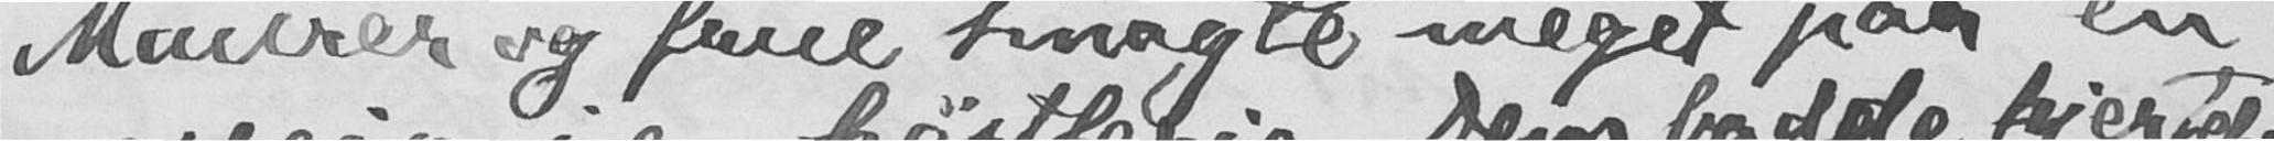Maurer og frue smagte meget paa en
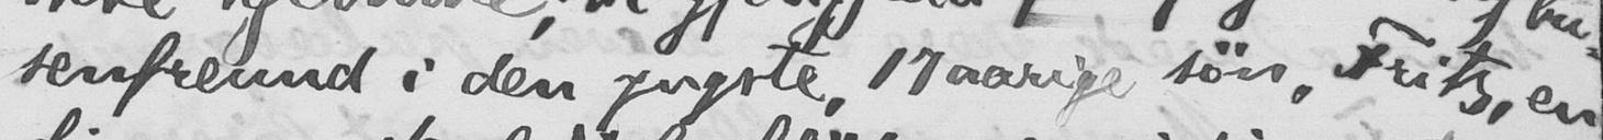senfreund i den yngste, 17 aarige søn, Fritz, en
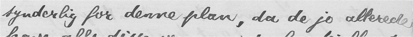synderlig for denne plan, da de jo allerede
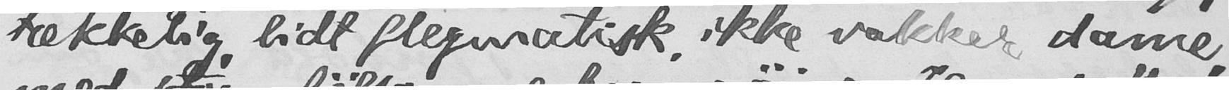tækkelig, lidt flegmatisk, ikke vakker dame,
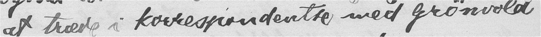at træde i korrespondentse med Grønvold
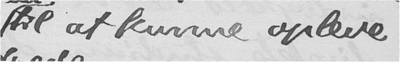til at kunne opleve
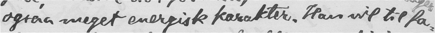ogsaa meget energisk karakter. Han vil til fa-
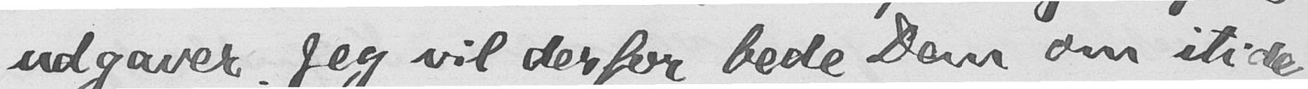udgaver. Jeg vil derfor bede Dem om itide
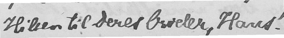Hilsen til Deres broder, Hans!
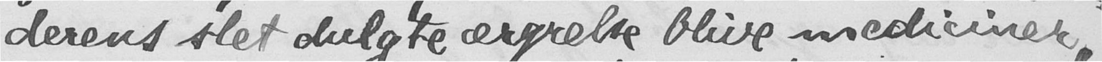derens slet dulgte ærgrelse blive mediciner,
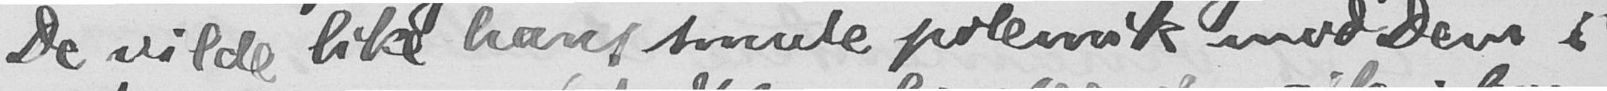De vilde likt hans smule polemik mod Dem i
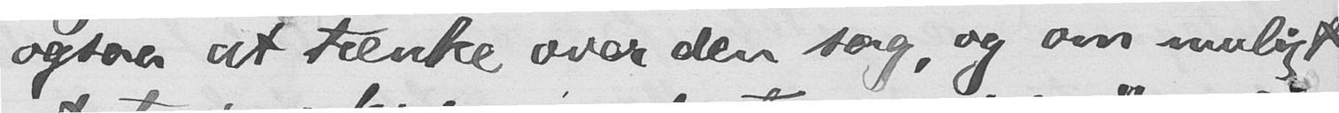ogsaa at tænke over den sag, og om muligt
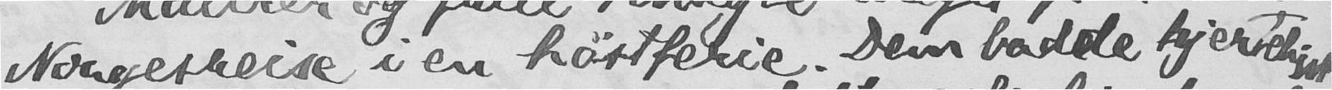Norgesreise i en høstferie. Dem bad de hjerteligst
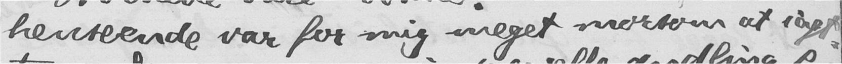henseende var for mig meget morsom at iagt-
